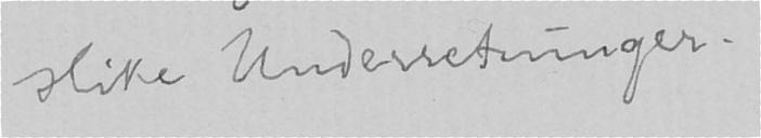slike Underretninger.
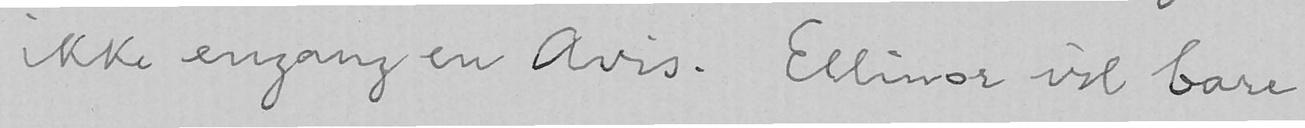ikke engang en Avis. Ellinor vil bare
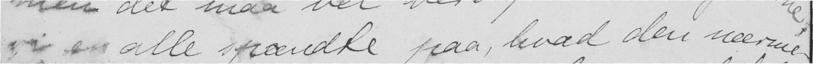vi er alle spendte paa, hvad den nærme
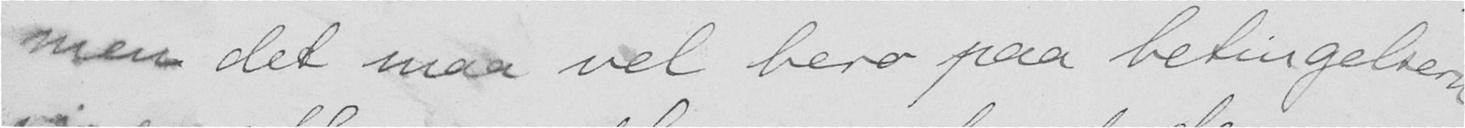men det maa vel bero paa betingelserne,
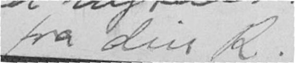fra din K.
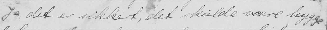Ja, det er sikkert, det skulde være hygge
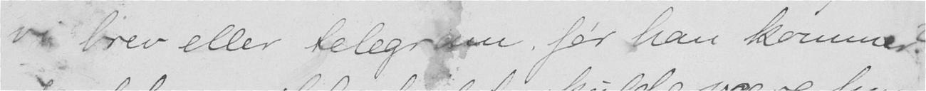vi brev eller telegram før han kommer?
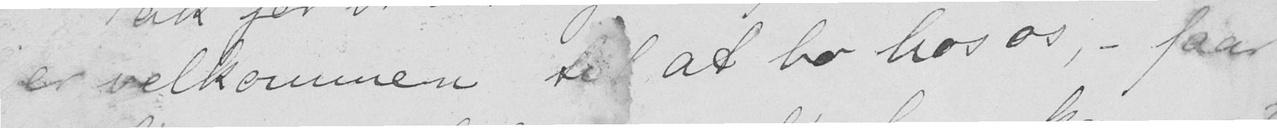er velkommen til at bo hos os, - faar
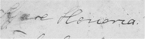Kjære Honoria!
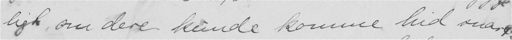ligt, om dere kunde komme hid snart,
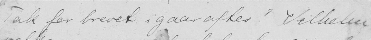Tak for brevet i gaar aftes! Vilhelm
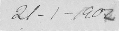21-1-1902
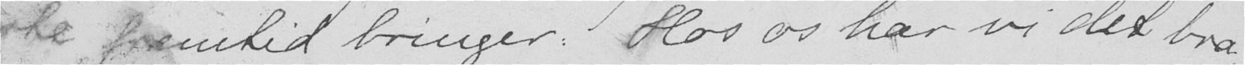ste fremtid bringer. Hos os har vi det bra
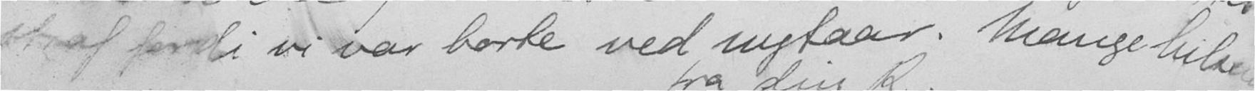straf fordi vi var borte ved nytaar. Mange hilsener
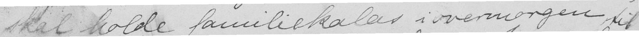skal holde familiekalas i overmorgen til
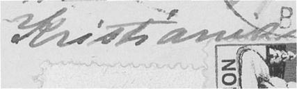Kristiania
